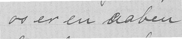os er en aaben
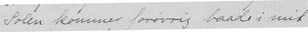Solen kommer forøvrig baade i mit
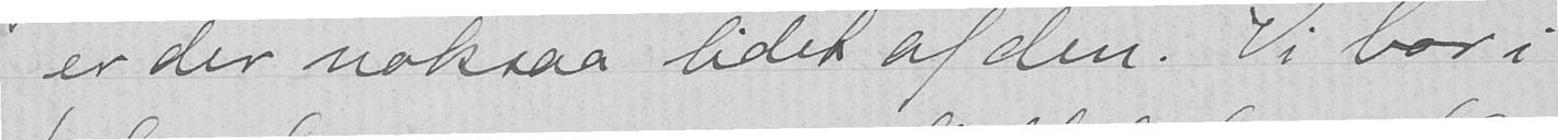er der noksaa lidet af den. Vi bor i
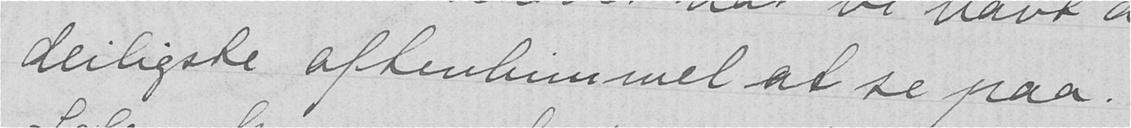deiligste aftenhimmel at se paa.
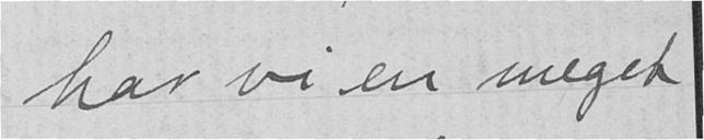har vi en meget
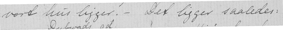vort hus ligger. - Det ligger saaledes:
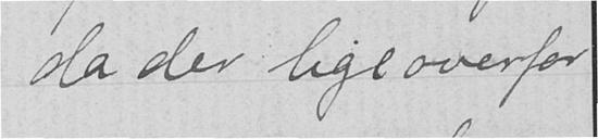da der lige overfor
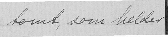tomt, som helder
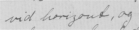vid horizont, og
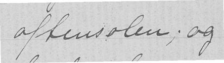aftensolen; og
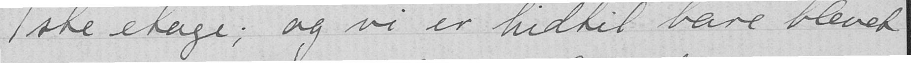1ste etage; og vi er hidtil bare blevet
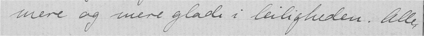mere og mere glade i leiligheden. Alle
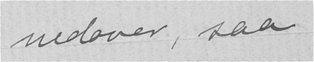nedover, saa
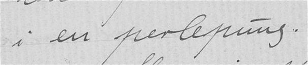i en perlepung.
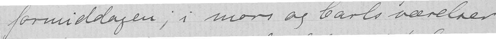formiddagen; i mors og Carls værelser
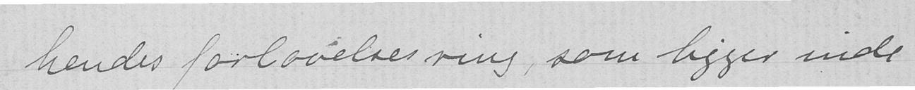hendes forlovelsesring, som ligger inde
